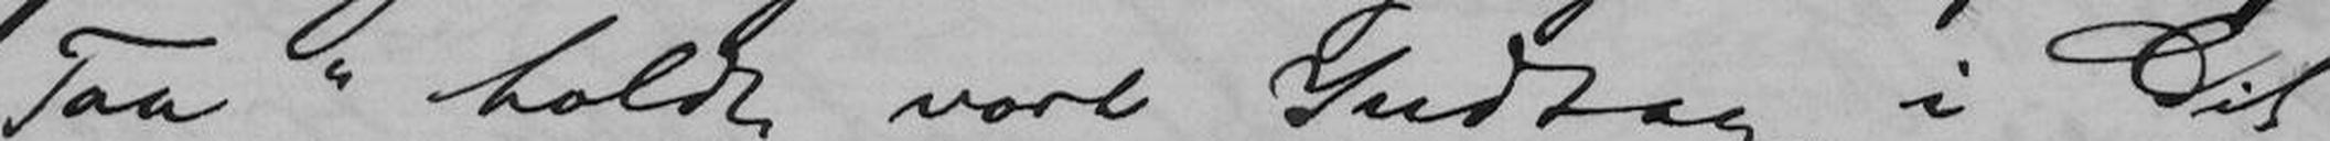Taa" holdt vort Indtog i Dit
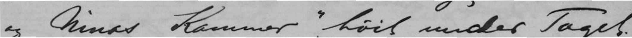og Ninas Kammer "høit under Taget".
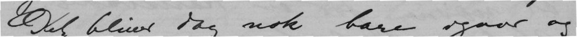Det bliver dog nok bare igaar og
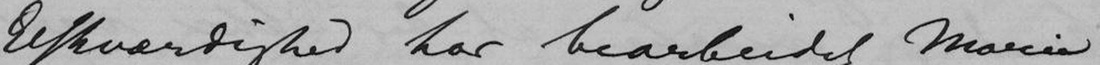Elskværdighed har bearbeidet Marie
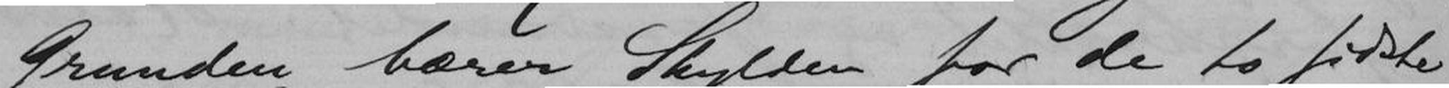Grunden bærer Skylden for de to sidste
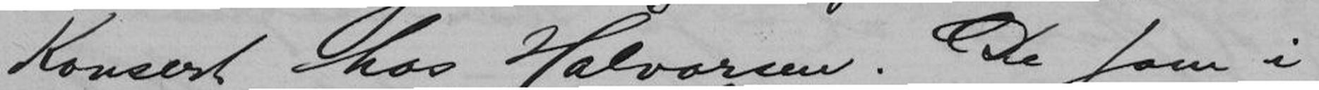Konsert hos Halvorsen. De som i
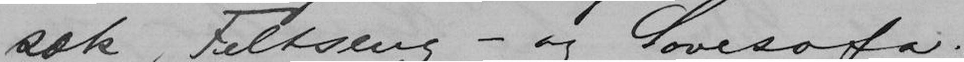sæk, Feltseng - og Sovesofa.
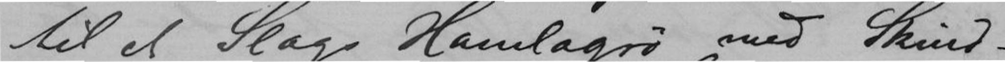til et Slags Hamlagrø med Skind-
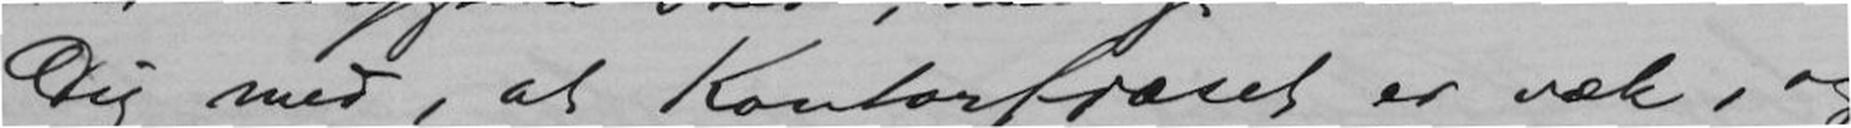Dig med, at Kontorfjæset er Væk, og
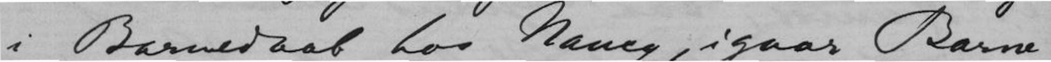i Barnedaab hos Nancy, igaar Barne-
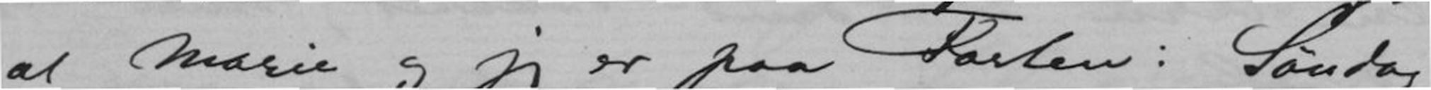at Marie og jeg er paa Farten: Søndag
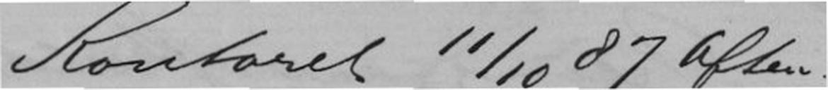Kontoret 11/10 87 Aften.
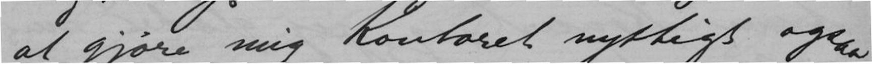at gjøre mig Kontoret nyttigt ogsaa
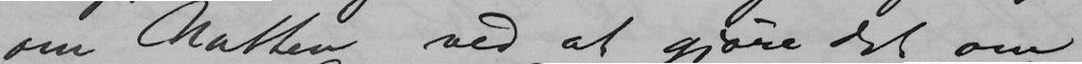om Natten ved at gjøre det om
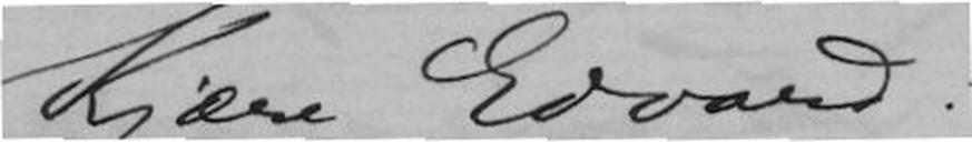Kjære Edvard!
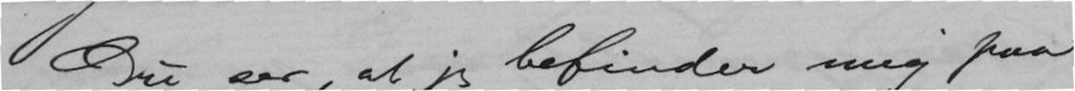Du ser, at jeg befinder mig paa
